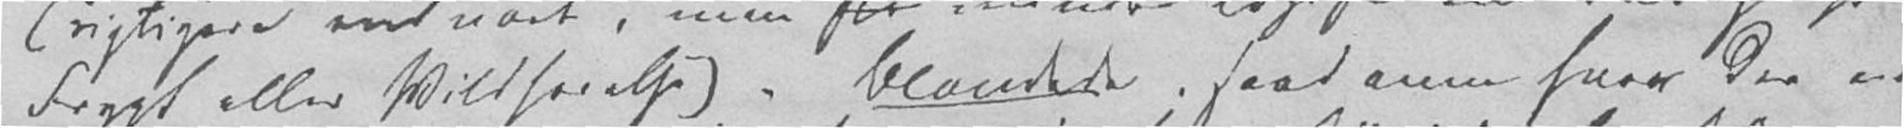Frygt eller Vildfarelse). Blandede, saadanne hvor der er
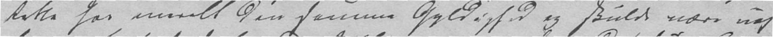Rette har overalt den samme Gyldighed og skulde være uaf-
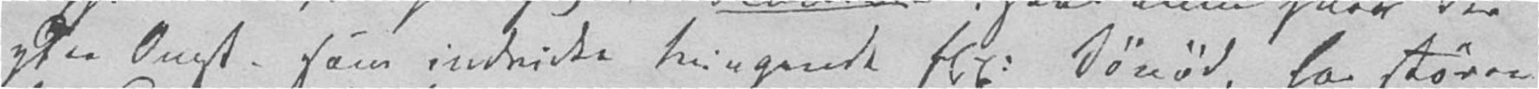ytre Omst. som indvirke tvingende fEx: Sønød, har større
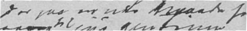der paa en Maade har
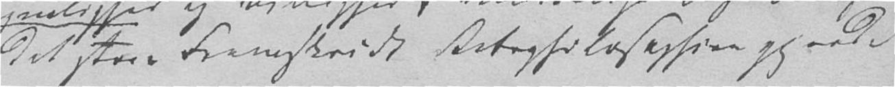det store Fremskridt Retsphilosophien gjorde
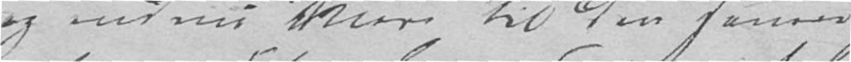og endnu Mere til den senere
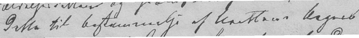Dette til Bestemmelse af Urettens Begreb
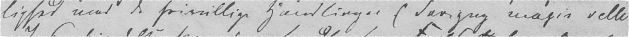Lighed med de frivillige Handlinger (Savigny mager alle).
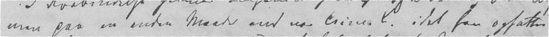men paa en anden Maade end vor Livse, idet han opfatter
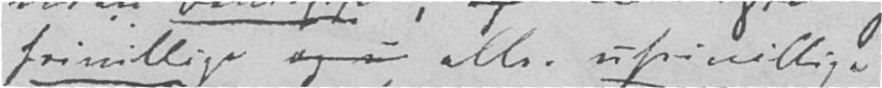frivillige og u eller ufrivillige
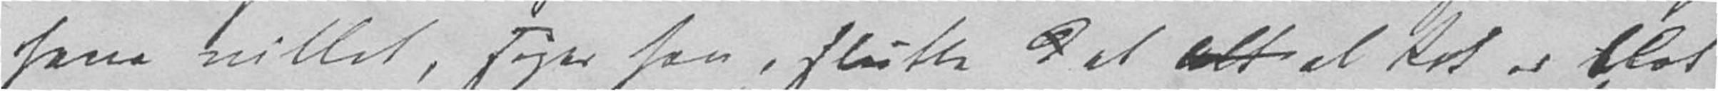have villet, siger han, slutte d at Alt al Ret er blot
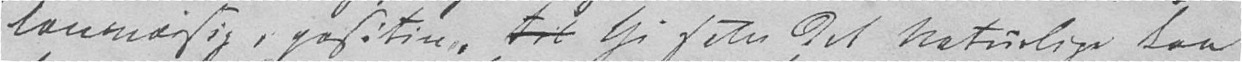Lovmæssig, positiv, til thi selv det Naturlige kan
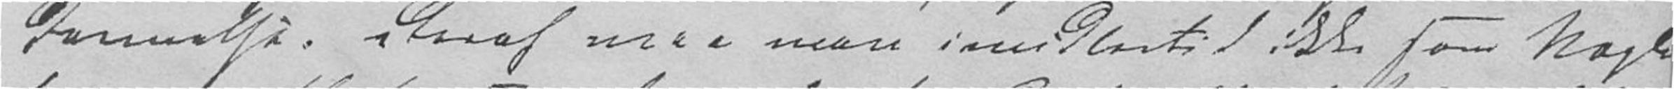Dannelse, Deraf maa man imidlertid ikke som Nogle
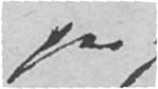paa
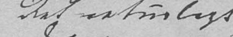Det naturligt
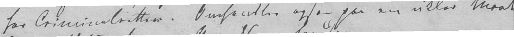for Criminalretten. Omhandler ogsaa paa en uklar Maade
